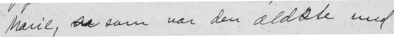Marie,  som var den ældste med
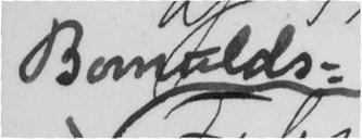Bomulds
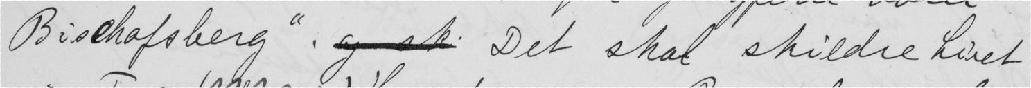Bischafsberg".  Det skal skildre Livet
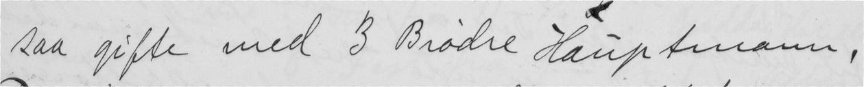saa gifte med 3 Brødre Hauptmann,
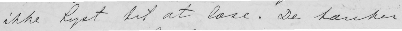ikke Lyst til at læse. De tænker
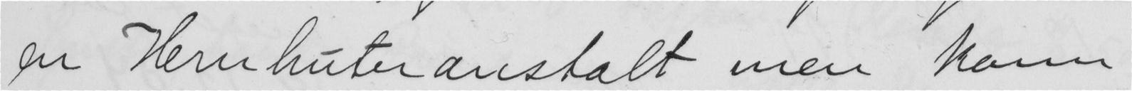en Hernhuteranstalt, men kom
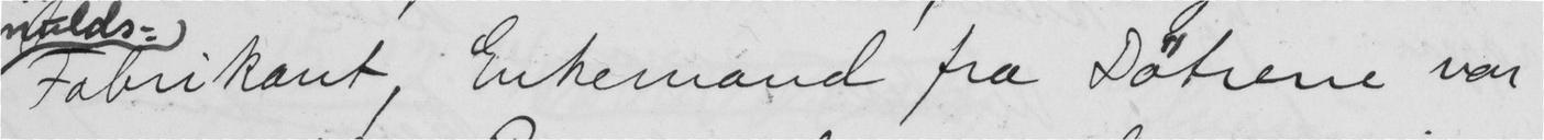Fabrikant, Enkemand fra Døtrene var
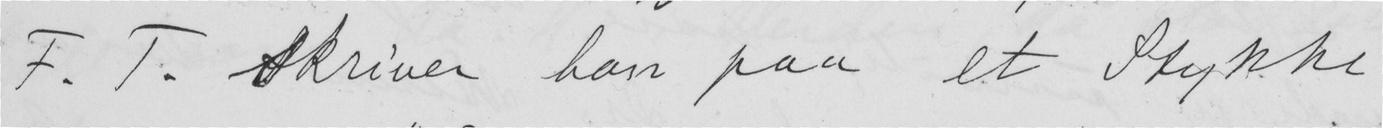F. T. skriver han paa et Stykke
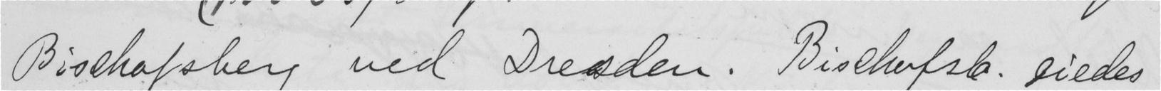Bischafsberg ved Dresden. Bischafsb: eiedes
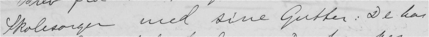Skolesorgen med sine Gutter: De har
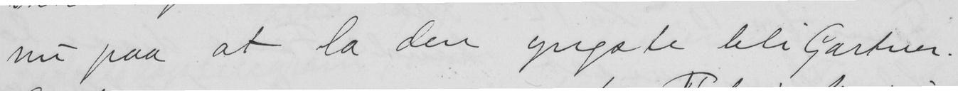nu paa at la den yngste bli Gartner.
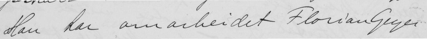Han tar om arbeidet Florian Geyer
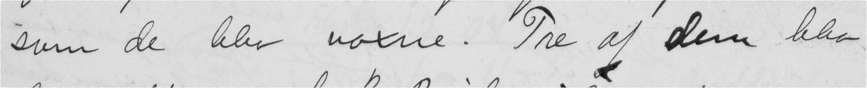sum de blev voxne. Tre af dem blev
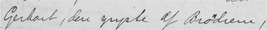Gerhart, den yngste af Brødrene,
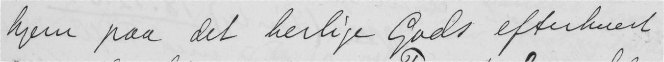hjem paa det herlige Gods efterhvert
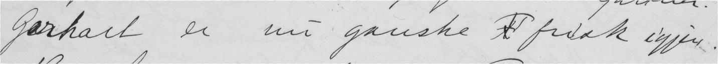Gerhart er nu ganske  frisk igjen.
